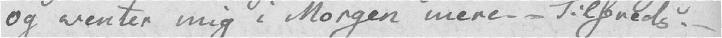og venter mig i Morgen mere – – Tilfreds. –
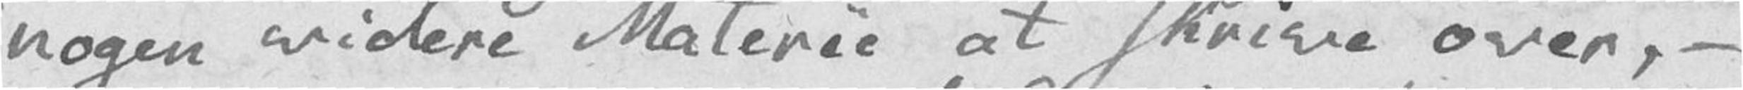nogen videre Materie at skrive over, –
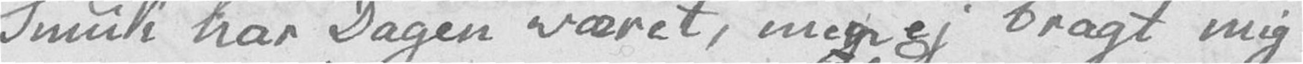Smuk har Dagen været, men ej bragt mig
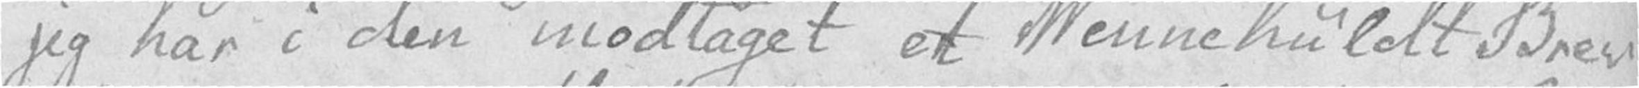jeg har i den modtaget et Vennehuldt Brev
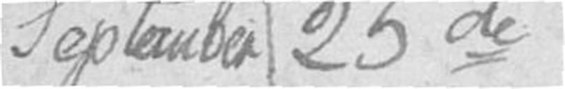September 25de
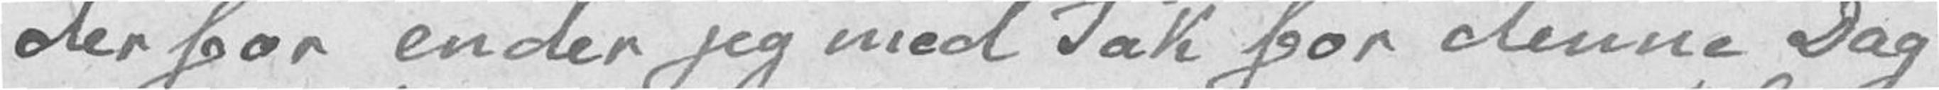derfor ender jeg med Tak for denne Dag
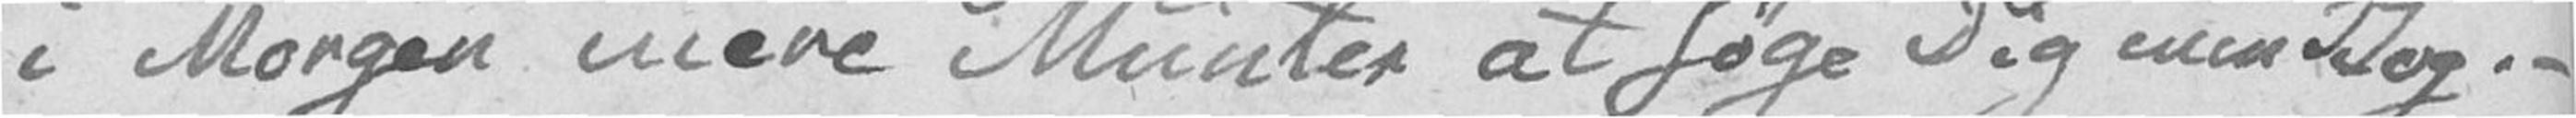i Morgen mere Munter at søge Dig min Bog. –
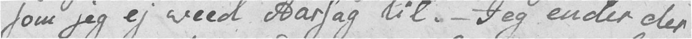som jeg ej veed Aarsag til. – Jeg ender der
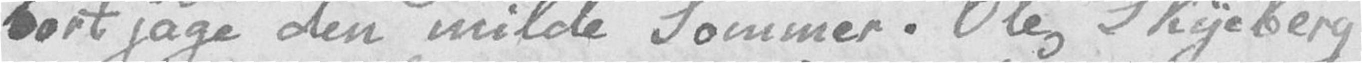bortjage den milde Sommer. Ole Skyeberg
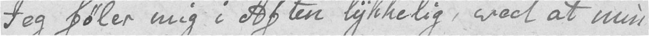Jeg føler mig i Aften lykkelig, ved at min
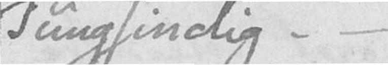Tungsindig. –
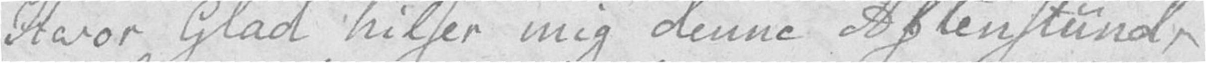Hvor Glad hilser mig denne Aftenstund,
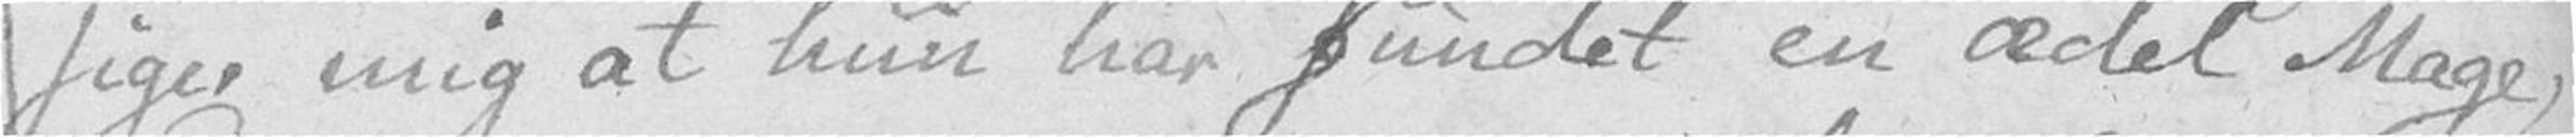siger mig at hun har fundet en ædel Mage,
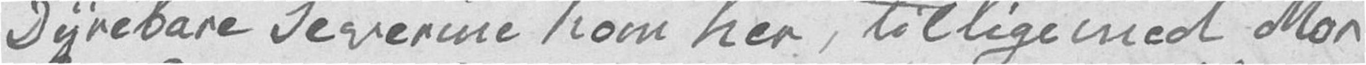Dyrebare Severine kom her, tilligemed Mor
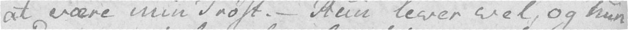at være min Trøst. – Hun lever vel og hun
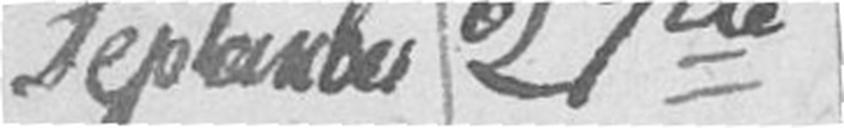September 27de
